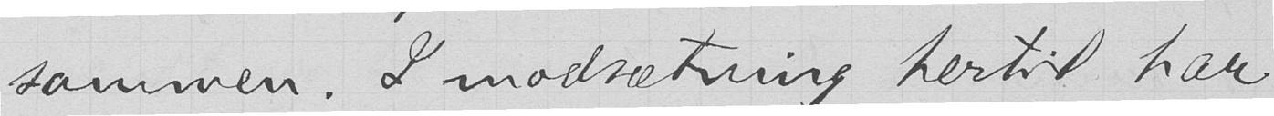sammen. I modsætning hertil har
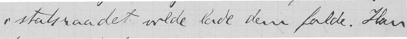i statsraadet vilde lade dem falde. Han
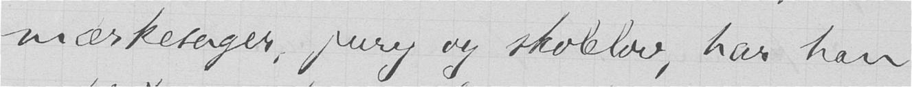mærkesager, jury og skolelov, har han
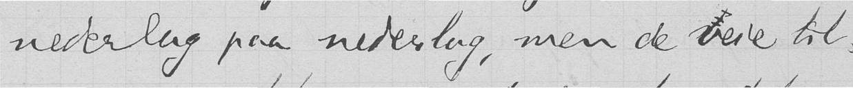nederlag paa nederlag, men de veie til-
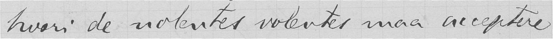hvori de nolentes volentes maa acceptere
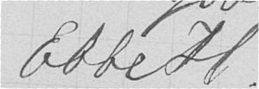Ebbe H.
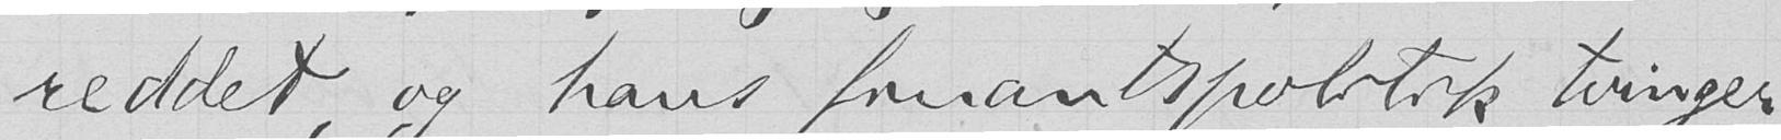reddet, og hans finantspolitik tvinger
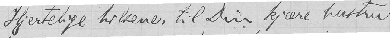Hjertelige hilsener til Din kjære hustru
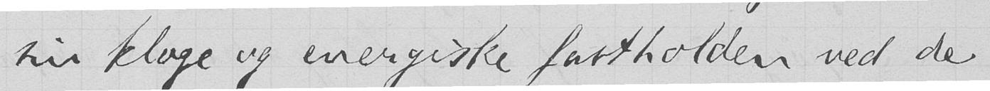sin kloge og energiske fastholden ved de
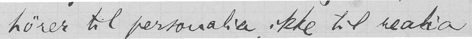hører til personalia, ikke til realia.
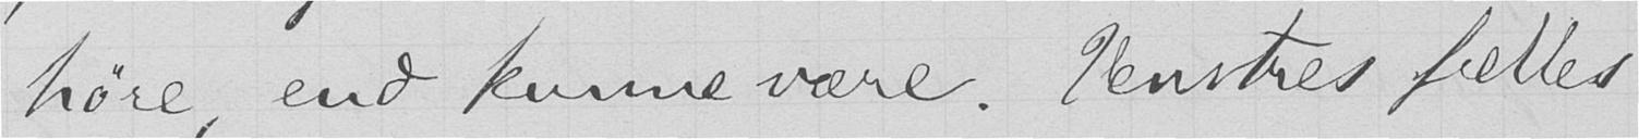høre, end kunne være. Venstres fælles
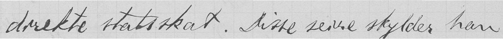direkte statsskat. Disse seire skylder han
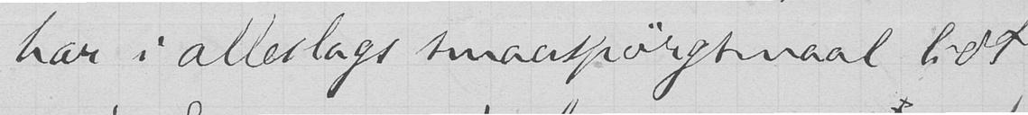har i alleslags smaaspørgsmaal lidt
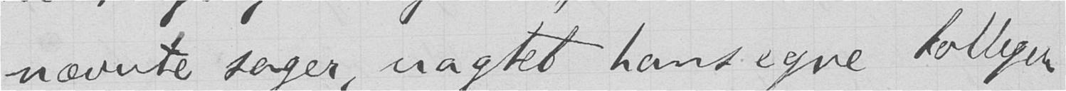nævnte sager, uagtet hans egne kolleger
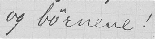og børnene!
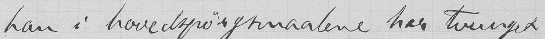han i hovedspørgsmaalene har tvinget
